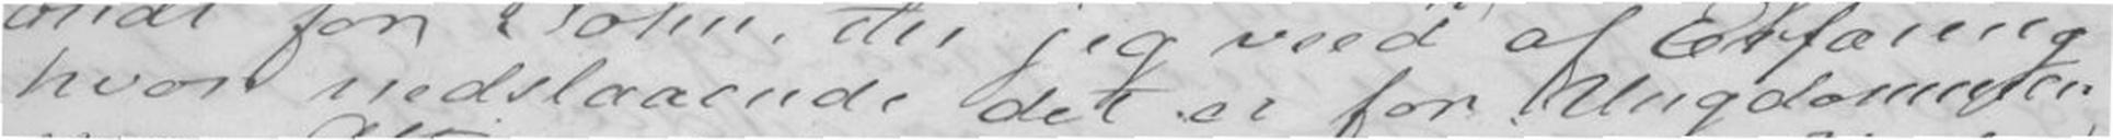hvor nedslaaende det er for Ungdommen
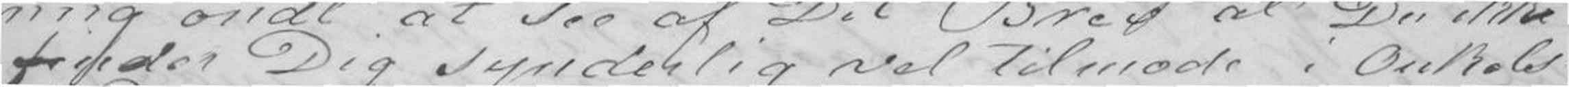finder Dig synderlig vel tilmode i Onkels
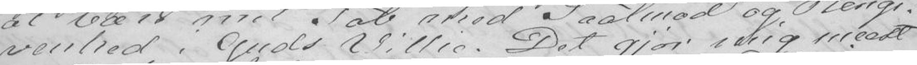venhed i Guds Villie. Det gjør mig meest
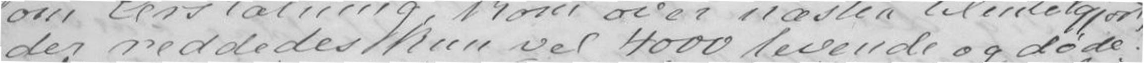der reddedes kun vel 4000 levende og døde.
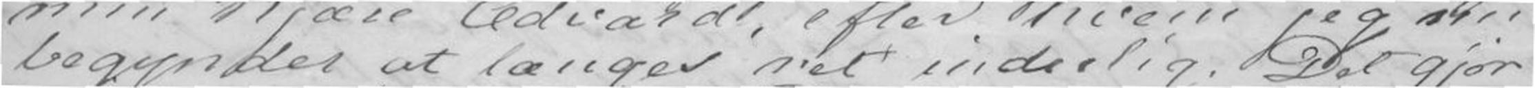begynder at længes ret inderlig. Det gjør
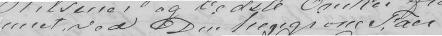met ved Din hengivne Faer
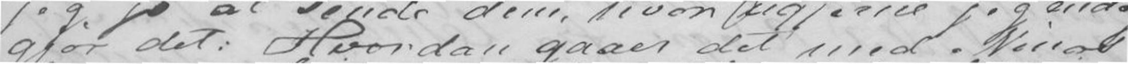gjør det: Hvordan gaaer det med Ninas
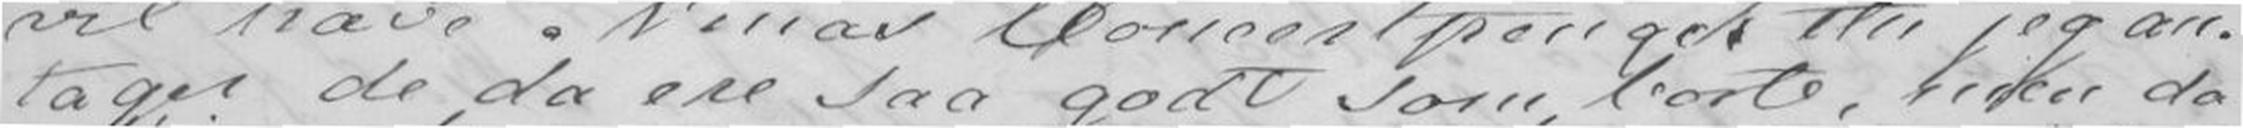tager de da ere saa godt som borte, men da
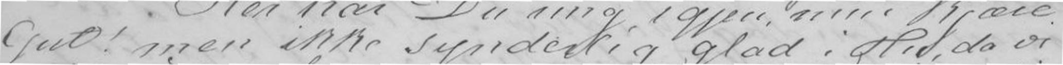Gut! men ikke synderlig glad i Hu, da vi
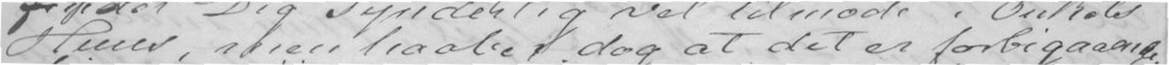Huus, men haaber dog at det er forbigaaende.
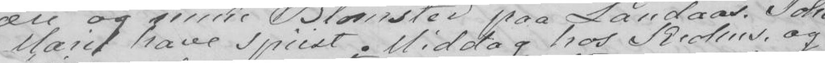Marie have spiist Middag hos Krohns, og
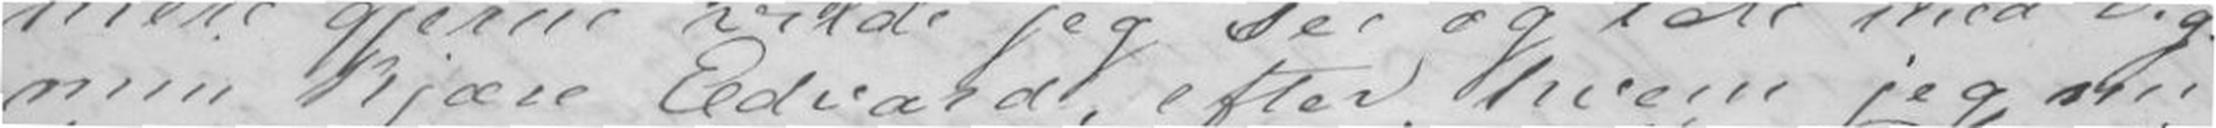min kjære Edvard, efter hvem jeg nu
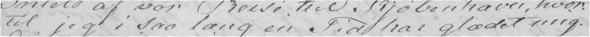til jeg i så lang en Tid har glædet mig.
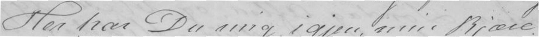Her har du mig igjen min kjære
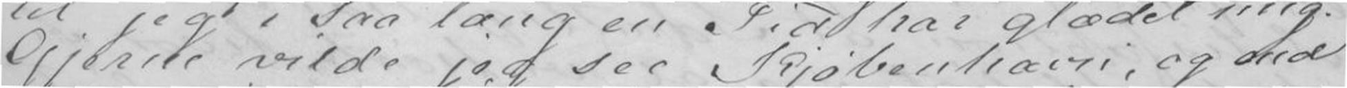Gjerne vilde jeg see Kjøbenhavn, og end
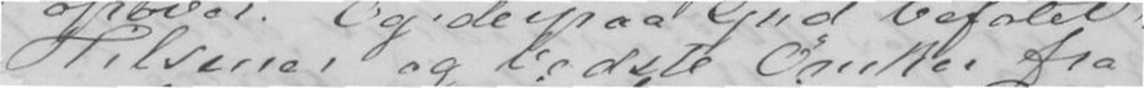Hilsener og bedste Ønsker fra
